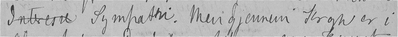Interesse Sympathi. Men gjennem Krogh er i
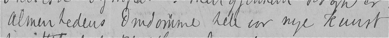Almenhedens Omdømme hele vor nye kunst,
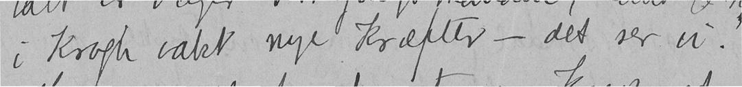i Krogh vakt nye Kræfter - det ser vi."
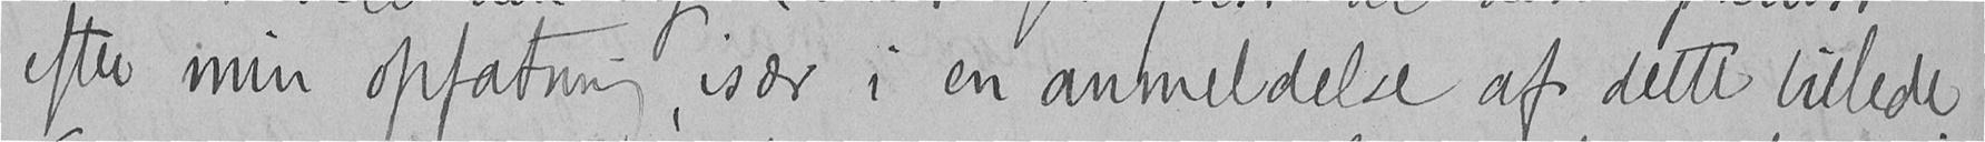efter min opfatning, især i en anmeldelse af dette billede.
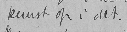kunst op i det.
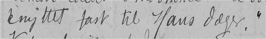knyttet fast til Hans Jæger."
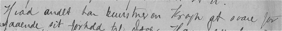Hvad andet har kunstneren Krogh at svare for
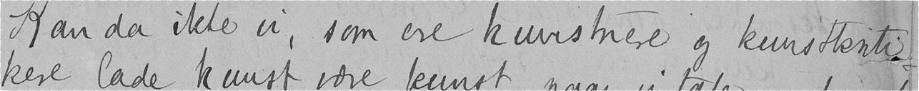Kan da ikke vi, som ere kunstnere og kunstkriti-
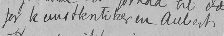for kunstkritikeren Aubert.
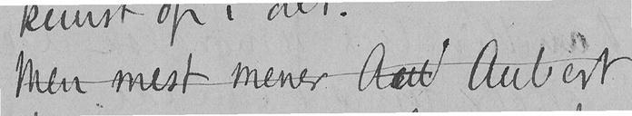Men mest mener And Aubert
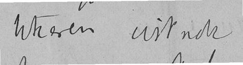tikeren vistnok
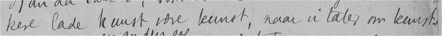kere lade kunst være kunst, naar vi taler om kunst.
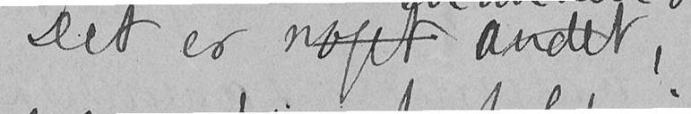Det er noget andet,
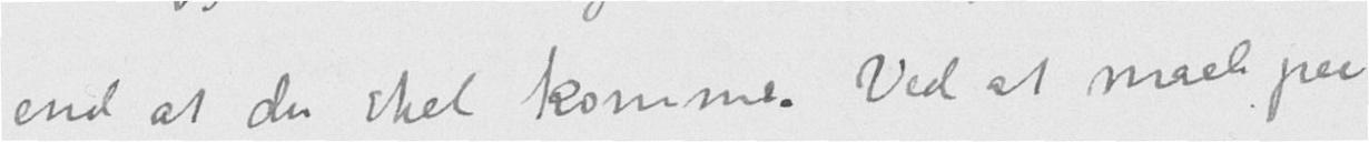end at du skal komme. Ved at maale paa
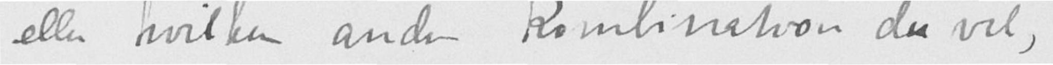eller hvilken anden Kombination du vil,
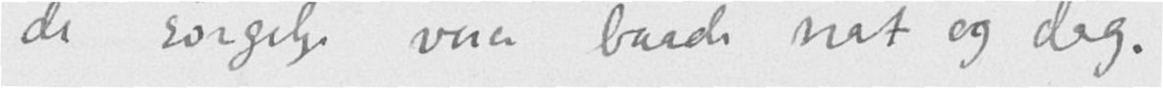de sørgelige veier baade nat og dag.
